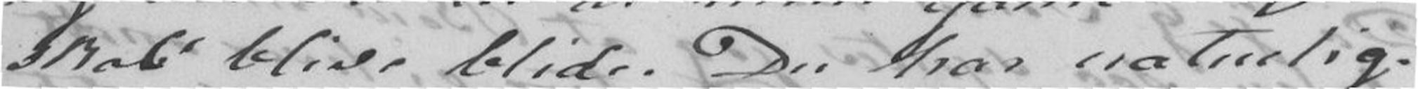skal blive blide. Du har naturlig-
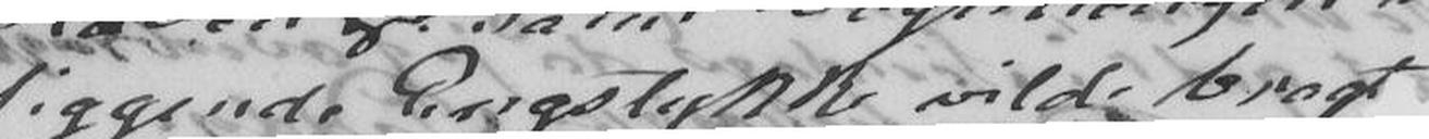liggende Engstykke vilde bragt
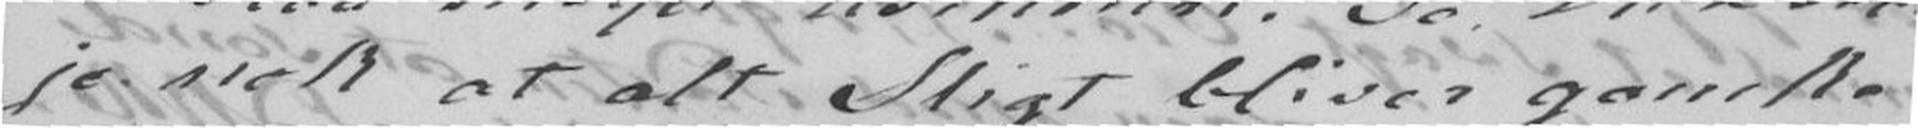jo nok at alt Sligt bliver ganske
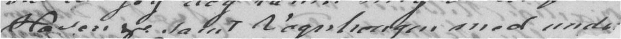Haven ogc. samt Vognhougen med uneBit borte
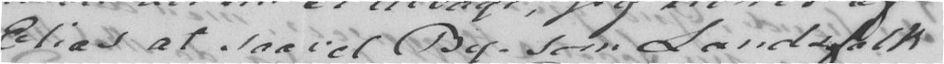Elias at saavel By- som Landsfolk
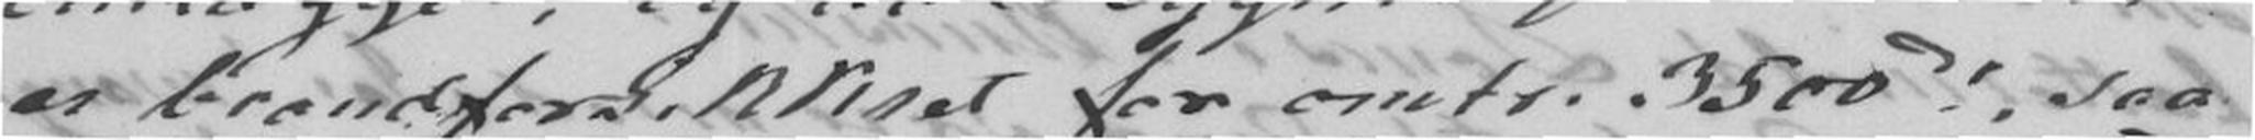er brandforsikkret for omtr. 3500do, saa
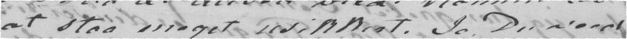at staa meget usikkert. Jo Du veed
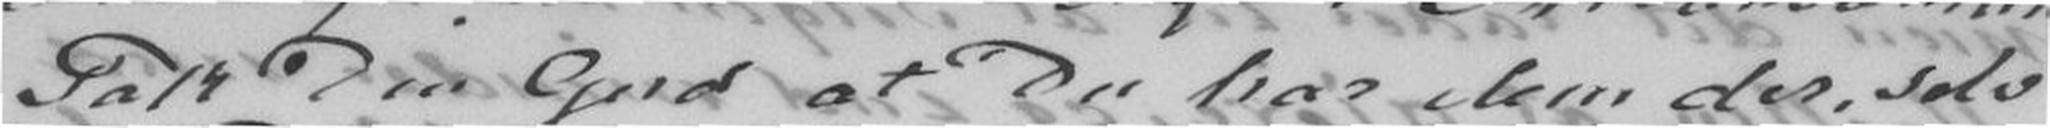Tak Din Gud at Du har dem der, selv
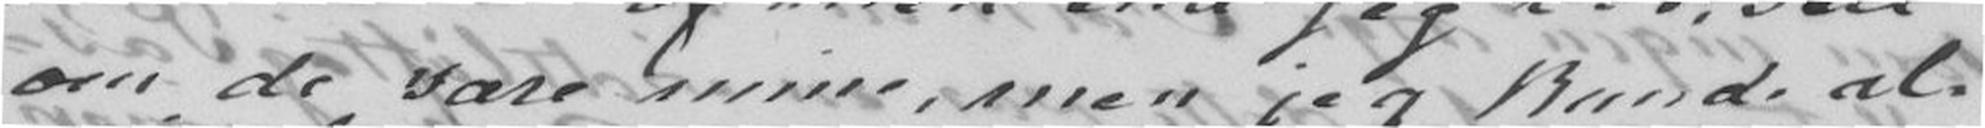om de være mine, men jeg kunde al-
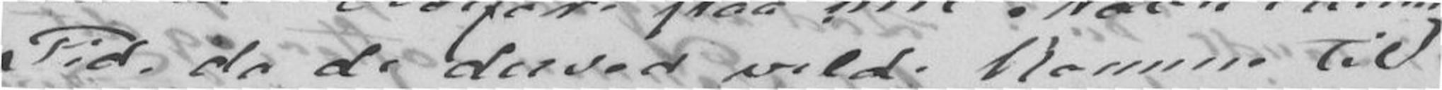Tid da de deved vilde komme til
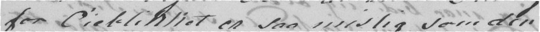for Øieblikket er saa mislig som den
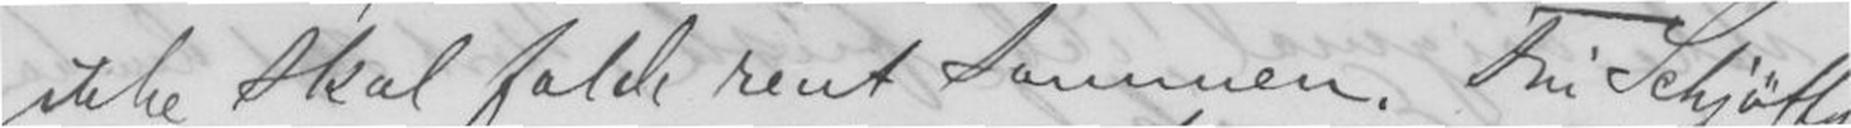ikke skal falde rent sammen. Fru Schjøtts
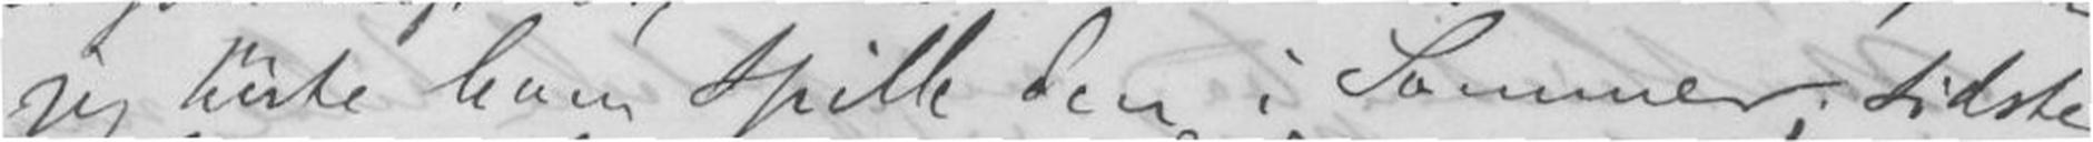jeg hørte ham spille den i Sommer; sidste
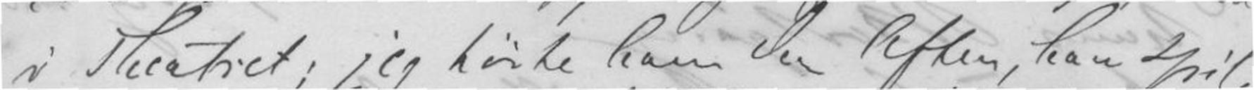i Theatret; jeg hørte ham den Aften, han spil-
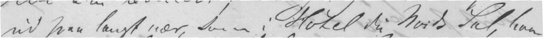ud paa langt nær, som i Hotel du Nords Sal, hvor
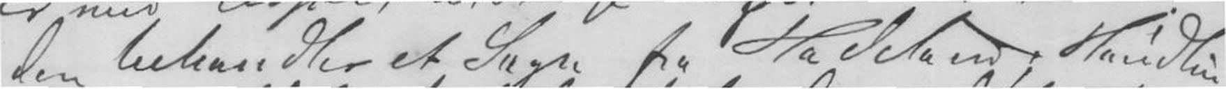den behandler et Sagn fra Hadeland; Handlin-
